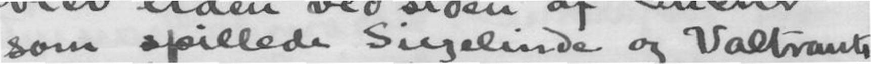som spillede Siegelinde og Valtraut,
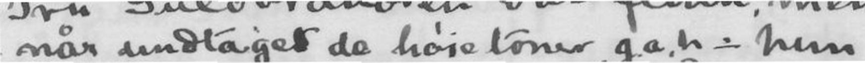- når undtaget da høie toner g a,h. - hun
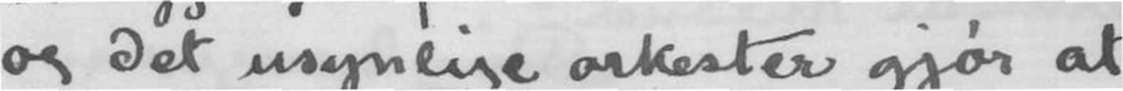og det usynlige orkester gjør at
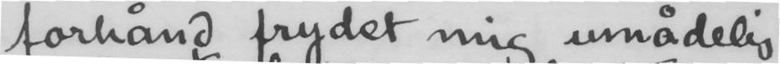forhånd frydet mig umådelig
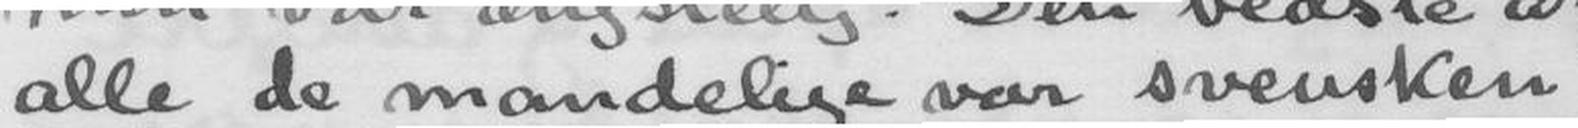alle de mandelige var svensken
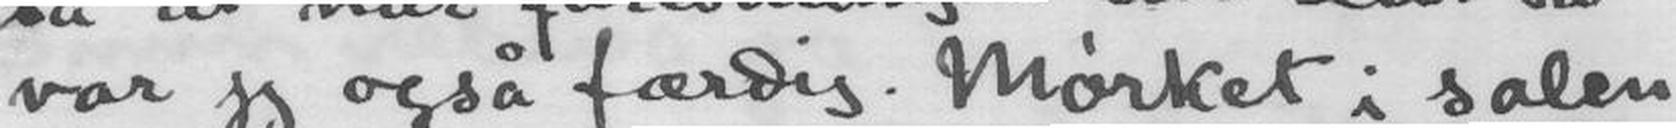var jeg også færdig. Mørket i salen
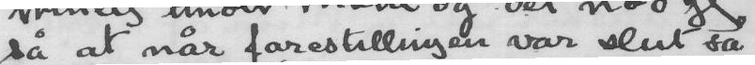så at når forestillingen var slut så
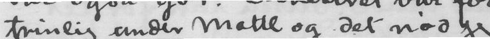trinlig under Motte og det nød jeg
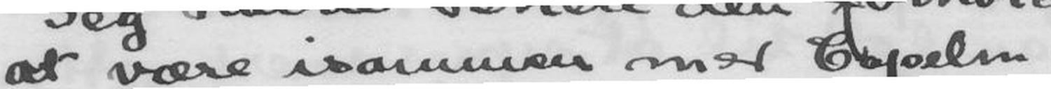at være isammen med Capelm
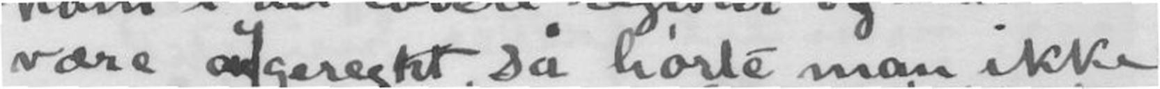være angereght, så hørte man ikke
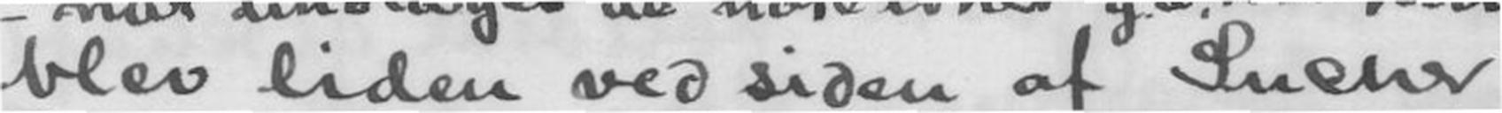blev liden ved siden af Suehr
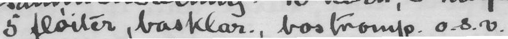5 fløiter, basklar., bastromp., o.s.v.,
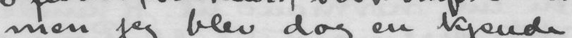men jeg blev dog en kjende
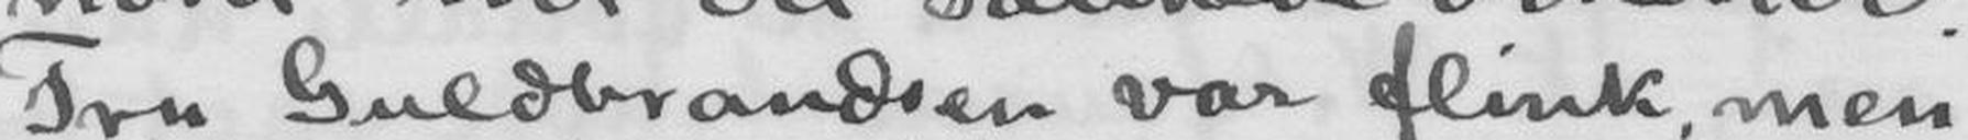Fru Guldbrandsen var flink, men
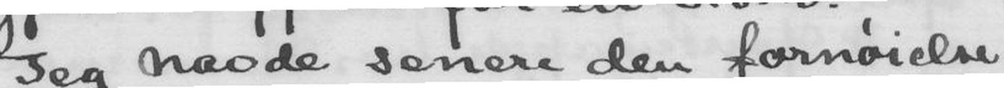Jeg havde senere den fornøielse
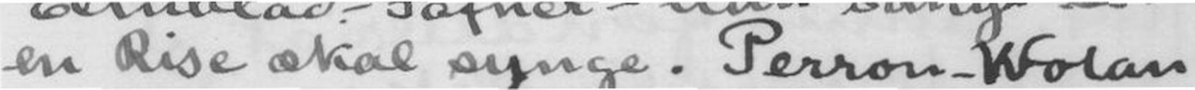en Rise skal synge. Perron-Wolan
